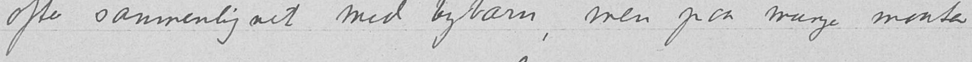ofte sammenlignet med bybarn, men paa mange maater
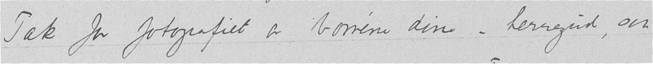Tak for fotografiet av børnene dine — herregud, saa
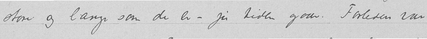store og lange som de er — ja tiden gaar. Forleden var
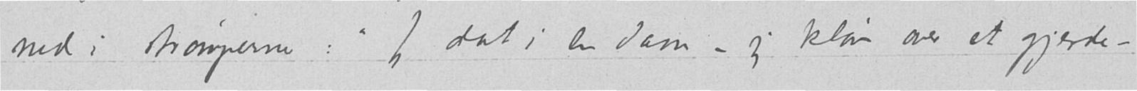ned i strømperne: "Jeg dat i en dam — jeg kløv over et gjerde —
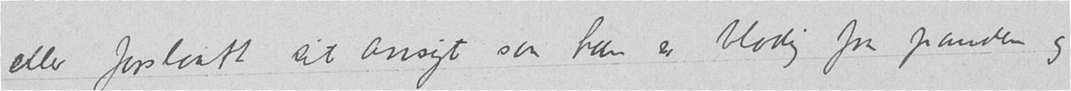eller forslått sit ansigt saa han er blodig fra panden og
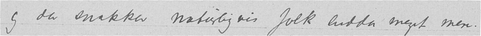og da snakker naturligvis folk endda meget mere.
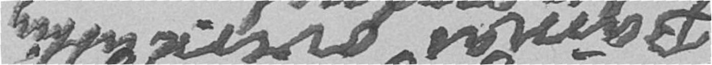Barnas overnatting
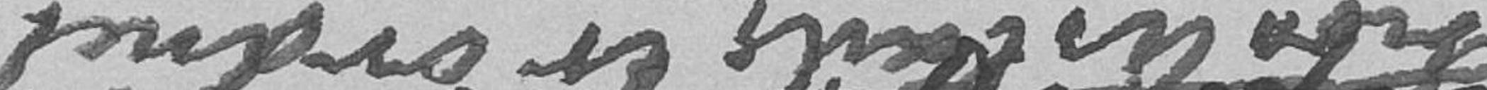hos Aslaug er ordnet
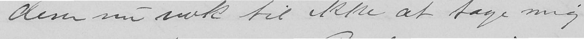dem nu nok til ikke at tage mig
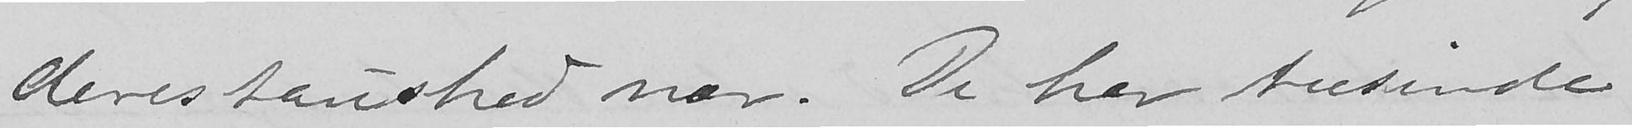deres taushed nær. De har tusinde
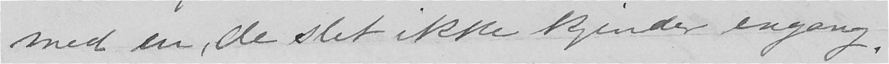med en, de slet ikke kjender engang.
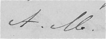A.M.
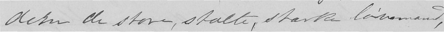dem de store, stolte, stærke løvemand,
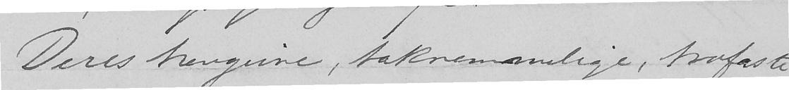Deres hengivne, taknemmelige, trofaste
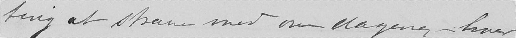ting at stræve med om dagene - hvor
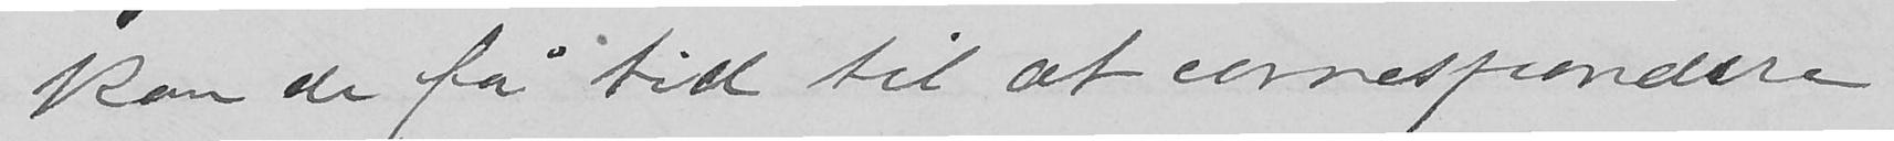kan de få tid til af correspondere
